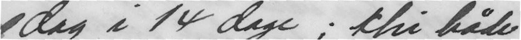dag i 14 dage; thi både
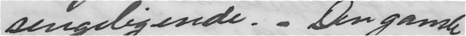sengeligende. - Den gamle
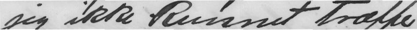jeg ikke kunnet træffe
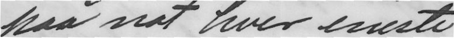paa nat hver eneste
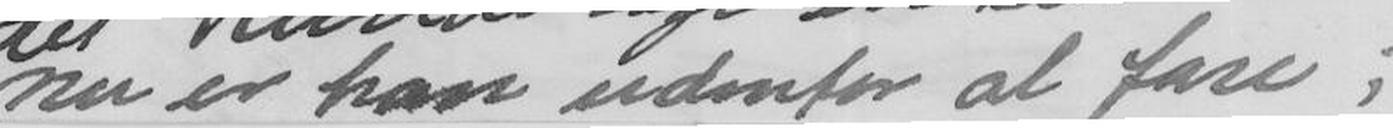Nu er han udenfor al fare;
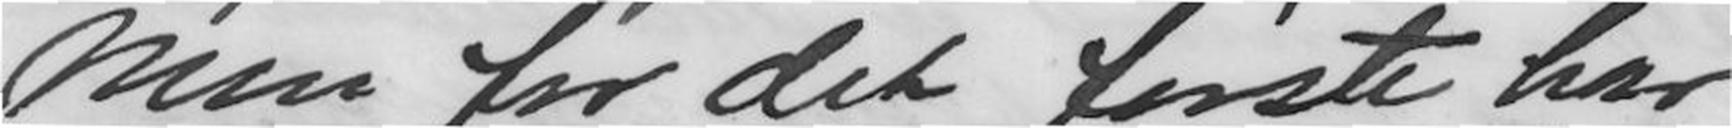Men for det første har
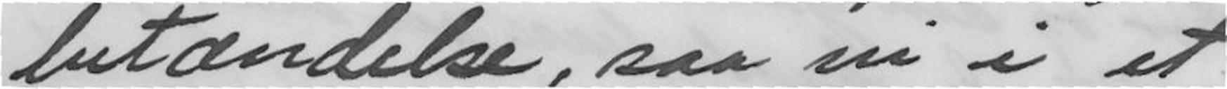betændelse, saa vi i et
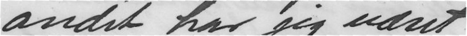andet har jeg været
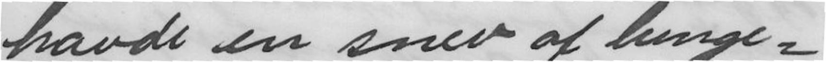havde en snev af lunge-
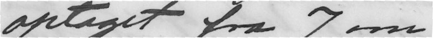optaget fra 7 om
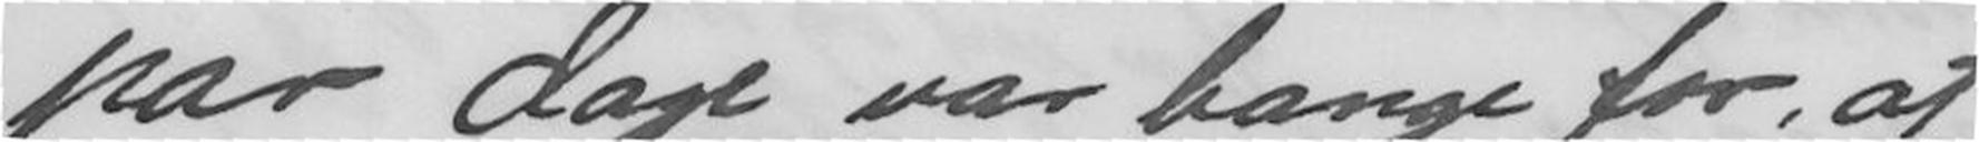par dage var bange for, at
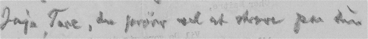Jaja Tore, du prøver vel at stræve paa din
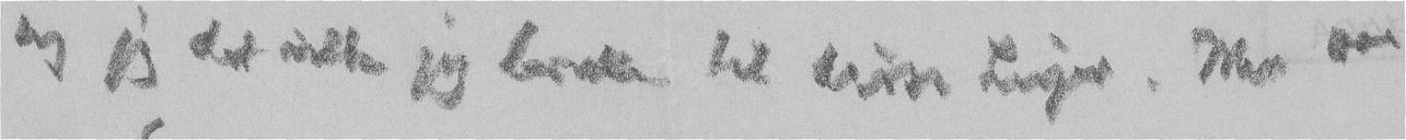og jeg det vilde jeg bruke til disse Linjer. Men nu
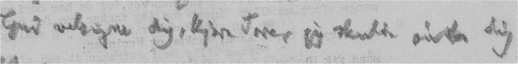Gud velsigne dig, kjære Tore, jeg skulde ønske dig
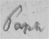Papa
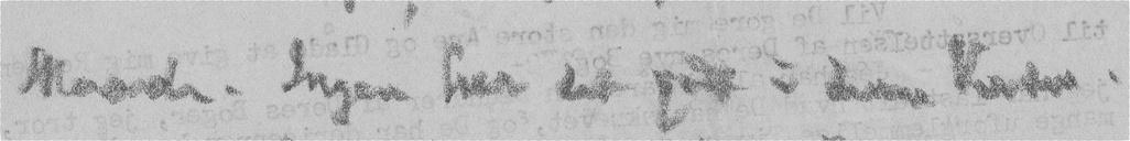Maate. Ingen har det godt i denne Verden.
